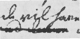de vil have
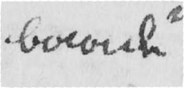baade
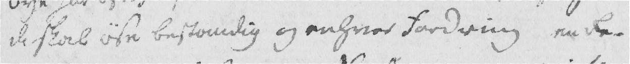de skal øse bestandig og enhver Fordring en Re-
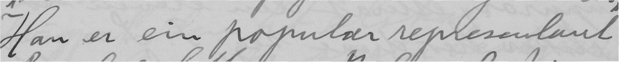Han er ein populær representant
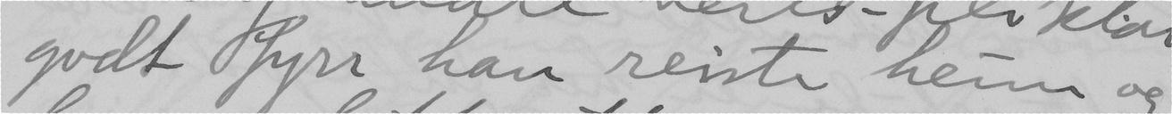godt fyrr han reiste heim og
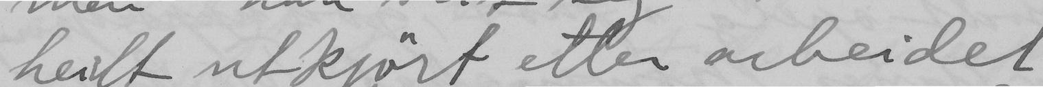heilt utkjört etter arbeidet
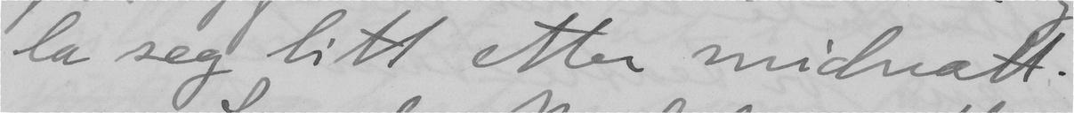la seg litt etter midnatt.
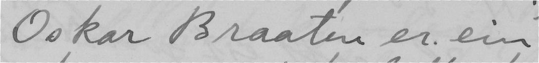Oskar Braaten er ein
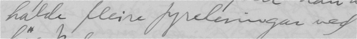halde fleire fyrelesingar ved
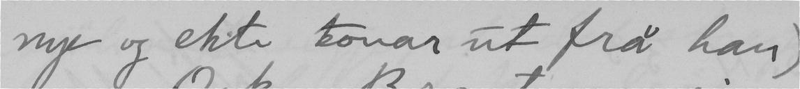nye og ekte tonar ut frå han.
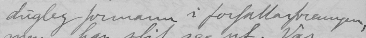dugleg formann i forfattarforeningen,
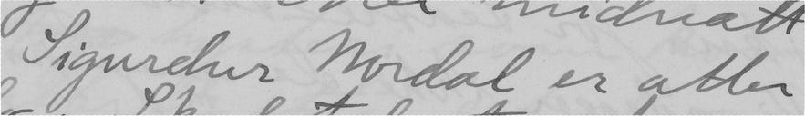Sigurdur Nordal er atter
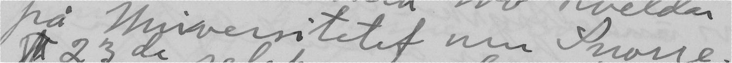på universitetet um Snorre.
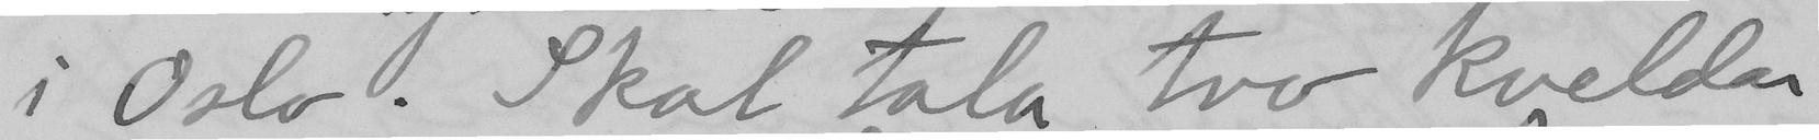i Oslo. Skal tala tvo kveldar
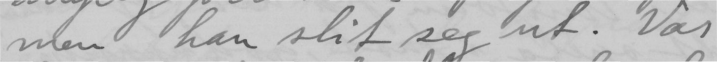men han slit seg ut. Var
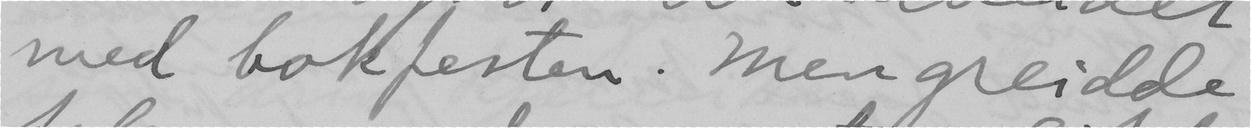med bokfesten. Men greidde
